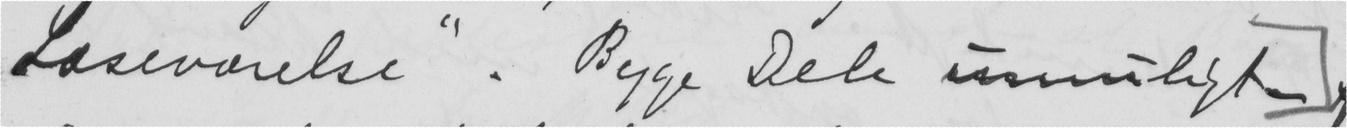Læseværelse". Begge Dele umuligt. Hag
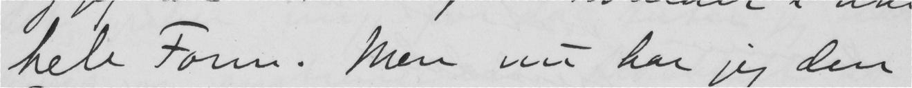hele Form. Men nu har jeg den
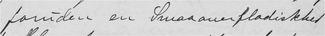foruden en Smaaoverfladiskhed
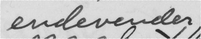endevender
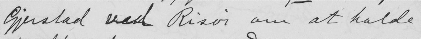Gjerstad ved Risør om at holde
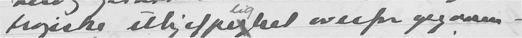tragiske uhjelpelighet overfor oppgaven -
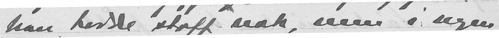han hadde stoff nok, men i ingen
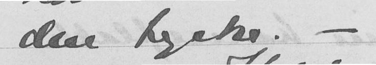den tyske. -
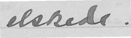elskede.
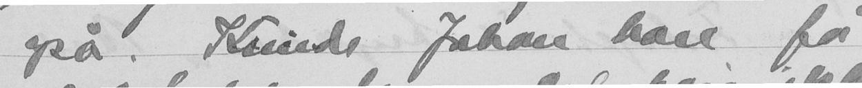også. Kunde Johan bare få
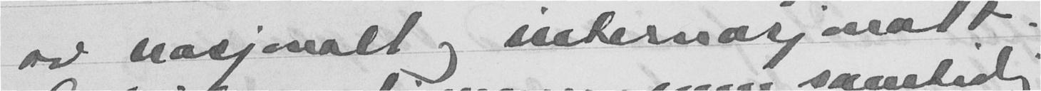av nasjonalt og internasjonalt.
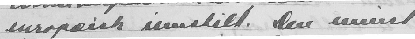europæisk innstilt. Den minst
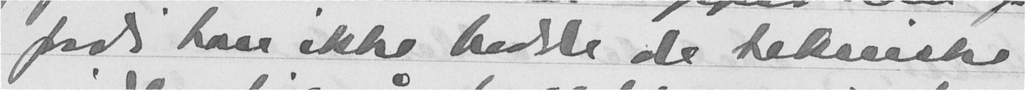fordi han ikke hadde de tekniske
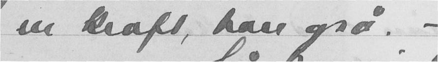en kraft, han også. -
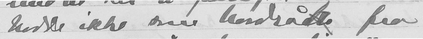hadde ikke som bordratte fra
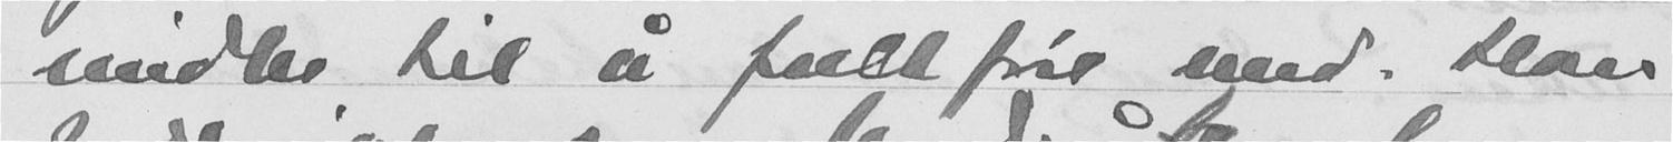midler til å fuillføre med. Han
